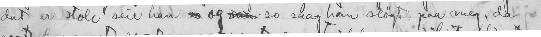dat er stole" seie han  og  so saag han støgt paa meg, da
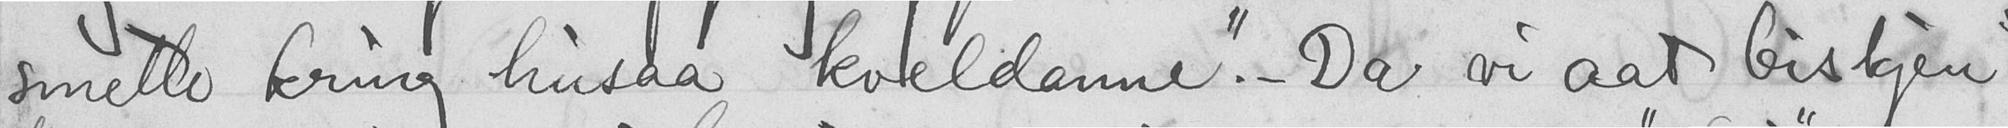smette kring husaa kveldanne". - Da vi aat biskjen
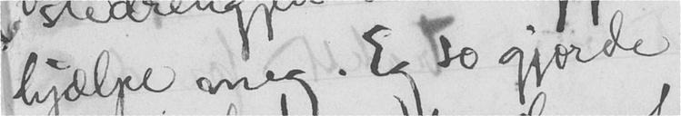hjælpe meg. Eg so gjorde
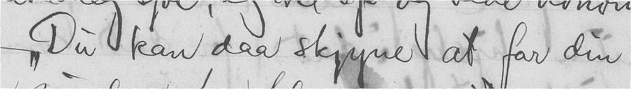"Du kan daa skjyne at far din
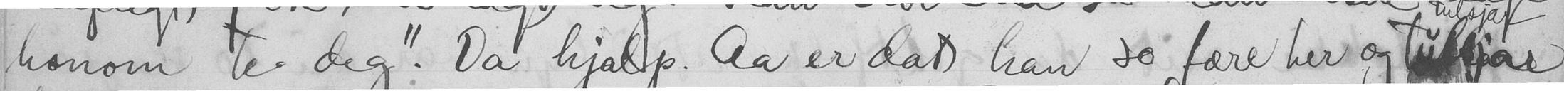honom te deg". Da hjalp. Aa er dat han so fære her og tuljar
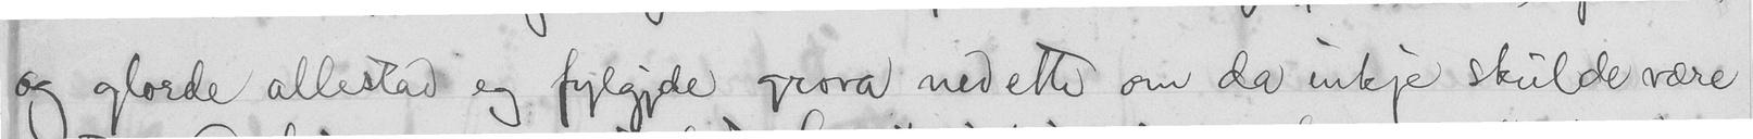og glorde allestad eg fylgjde grova ned ette om da inkje skulde være
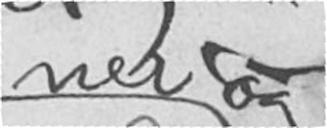ner og
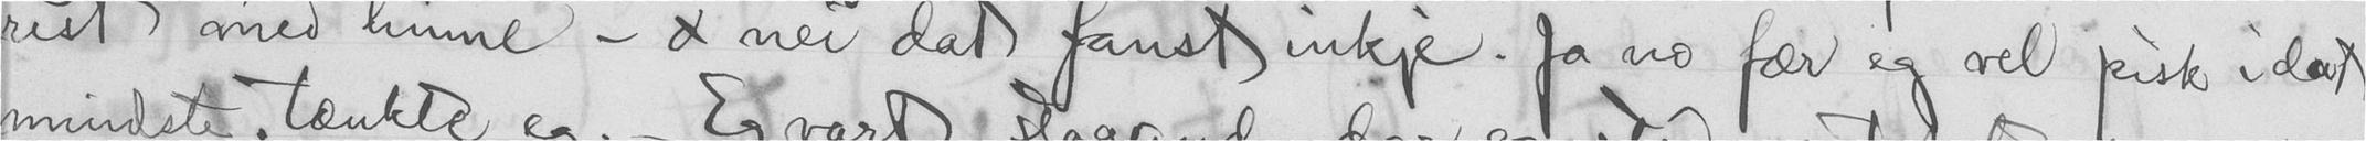rest med hinne -  nei dat fanst inkje. Ja no fær eg vel pisk i dat.
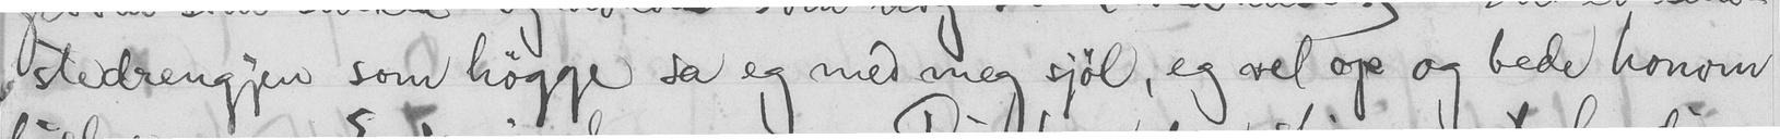stedrengjen som høgge sa eg med meg sjøl, eg vel op og bede honom
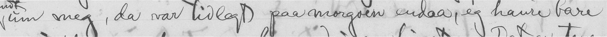um meg, da var tidlegt paa morgoen endaa, eg haure bare
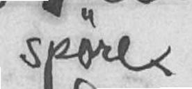spøre
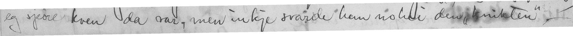eg spøre kven da var, men inkje svarde han nokei den "knikten".
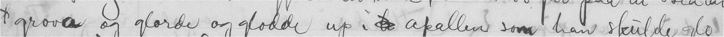grova og glorde og glodde up i  apallen som han skulde glo
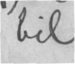til
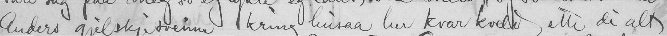Anders gjelskjesveime krine husaa her kvar kvelt, ette di alt
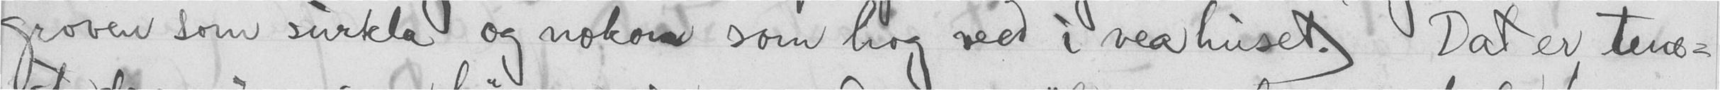groven som surkla og nokon som hog veed i veahuset. Dat er tene-
